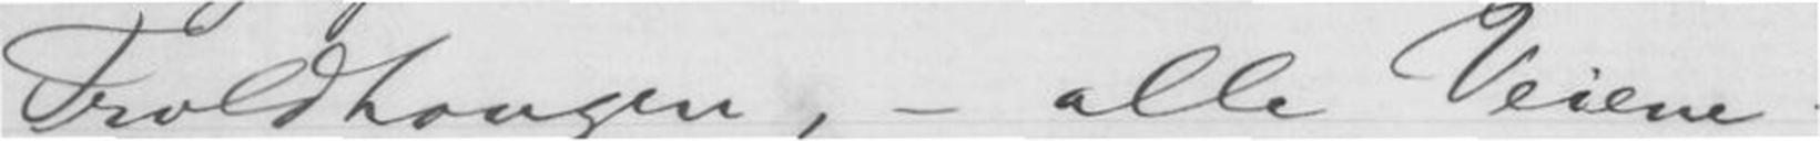Troldhaugen, - alle Veiene.
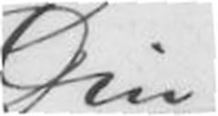Din
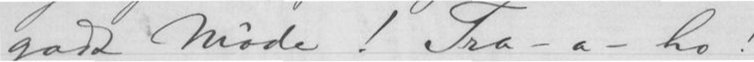godt Møde! Tra-a-ho!
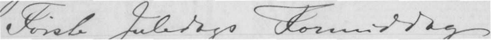Første Juledags Formiddag
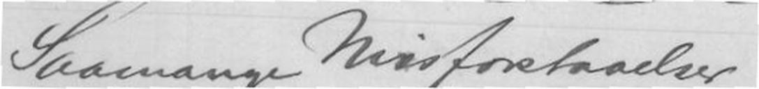Saamange Misforstaaelser
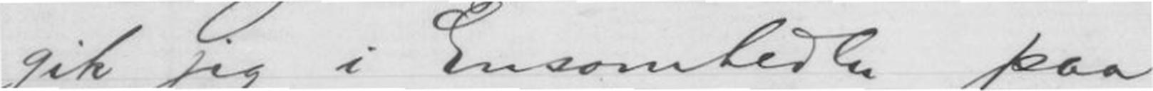gik jeg i Ensomheden paa
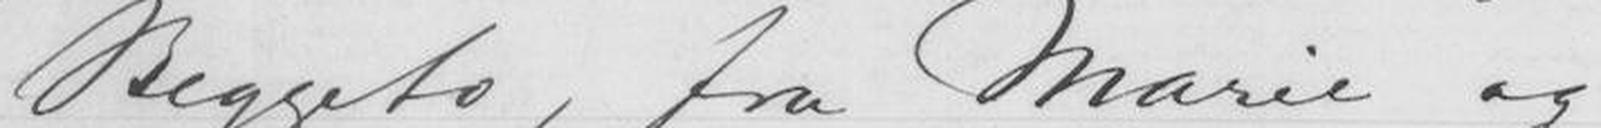Beggeto, fra Marie og
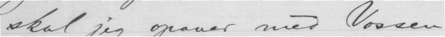skal jeg opover med Vossen,
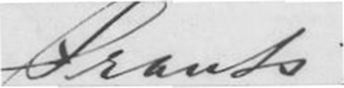Frants,
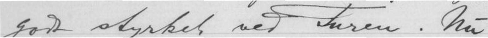godt styrket ved Turen. Nu
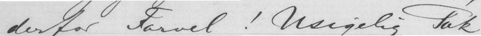derfor Farvel! Usigelig Tak
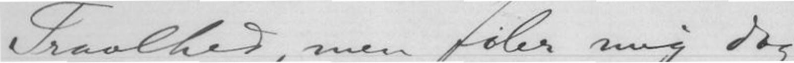Travlhed, men føler mig dog
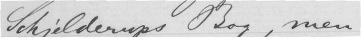Schjelderups Bog, men
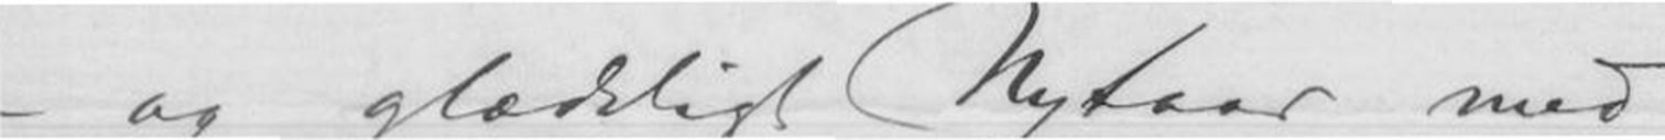- og glædeligt Nytaar med
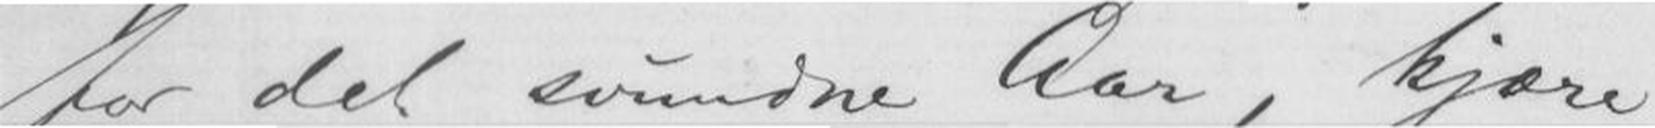for det svundne Aar, kjære
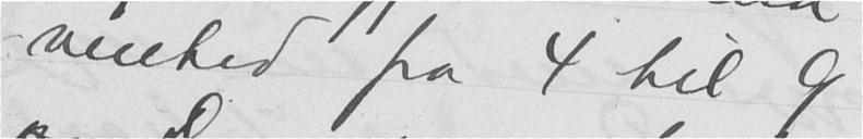vented fra 4 til 9
